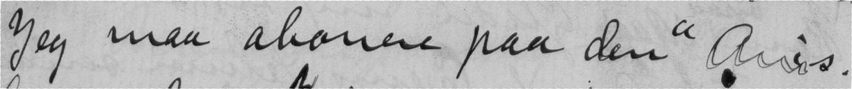Jeg maa abonere paa den "avis.
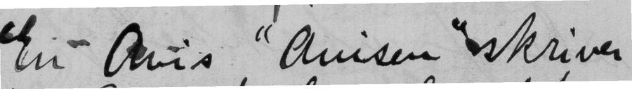en avis "Avisen" skriver
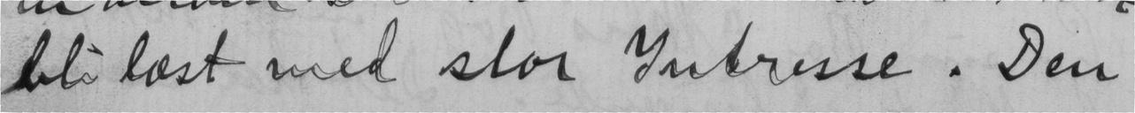bli læst med stor interesse. Den
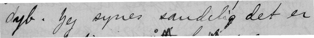dyb. Jeg synes sandelig det er
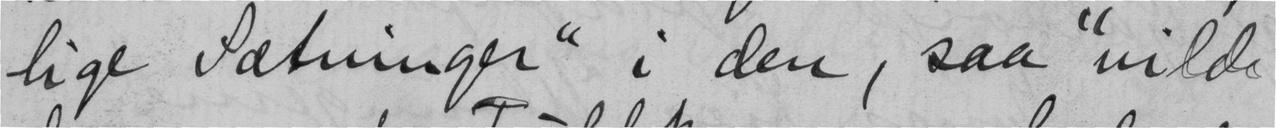lige Sætninger" i den, saa "vilde
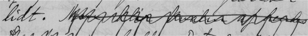lidt.
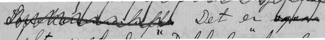Det er
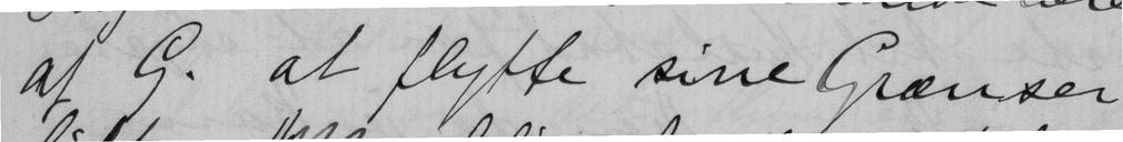af G. at flytte sine Grænser
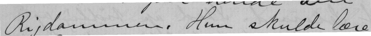Rigdommen. Hun skulde lære
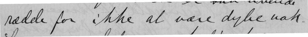rædde for ikke at være dybe nok.
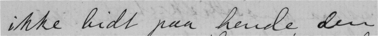ikke bidt paa hende den
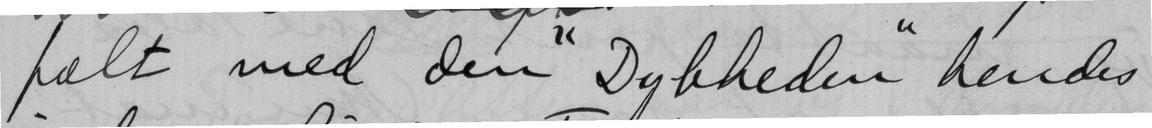fælt med den "Dybheden" hendes
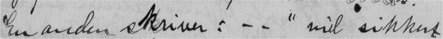En anden skriver: - "vil sikkert
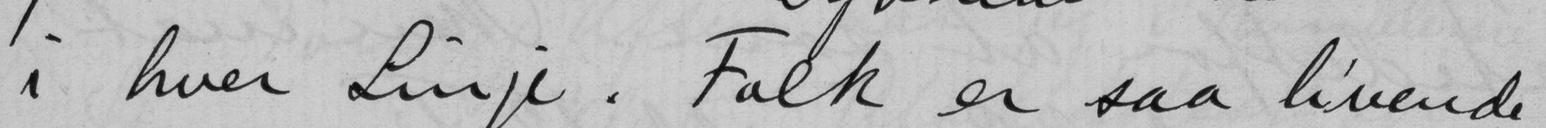i hver Linje. Folk er saa livende
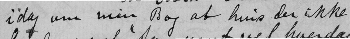i dag om min Bog at hvis den ikke
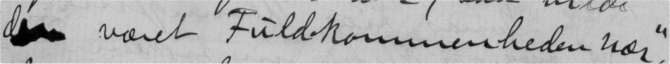været Fuldkommenheden nær".
